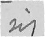sig
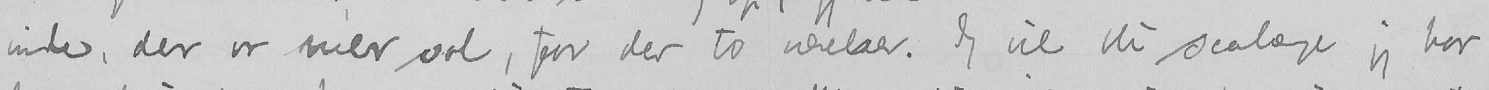inde, der er mer sol, for der to værelser. Jeg vil bli saalænge jeg har
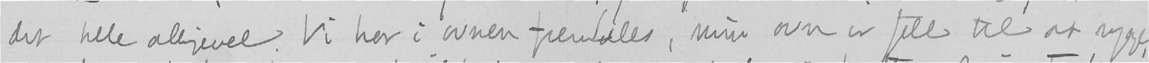det hele alligevel. Vi har i ovnen fremdeles, min ovn er ful til at ryge,
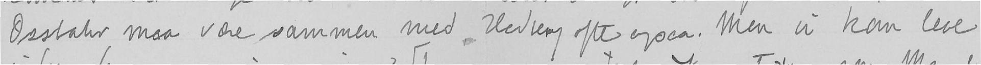Ossbahr maa være sammen med Hedberg ofte ogsaa. Men vi kan leve
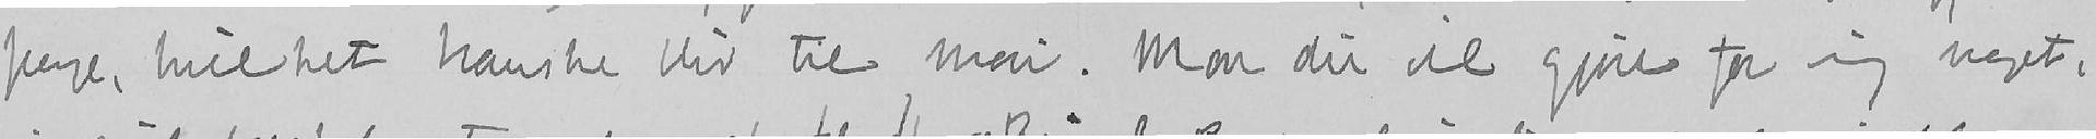penge, hvilket kanske blir til mai. Mon du vil gjøre for mig noget,
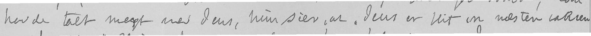havde talt meget med Jens, hun sier, at «Jens er blit en næsten voksen
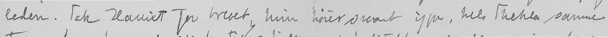leden. Tak Harriet for brevet, hun hører snart igjen, hils Thekla samme
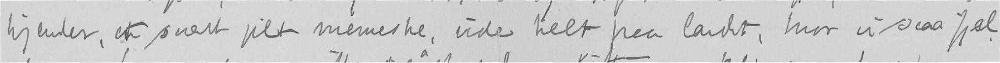kjender, et svært jilt menneske, ude helt paa landet, hvor vi saa fjel-
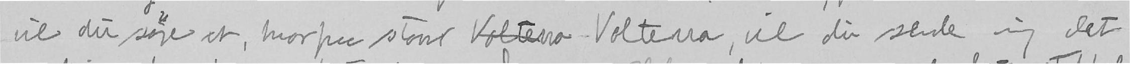vil du søge et, hvorpaa staar Voltera Volterra, vil du sende mig det
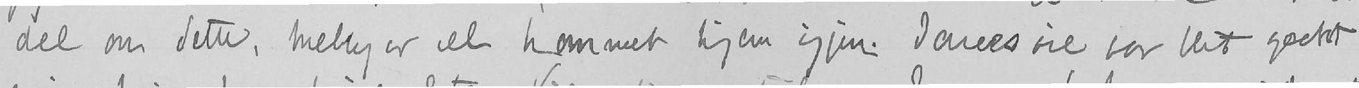del om dette, Melby er vel kommet hjem igjen. Jonas Lie har blit godt
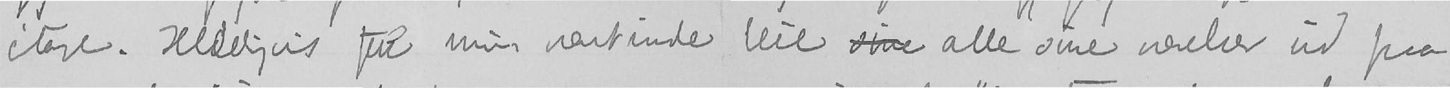étage. Heldigvis fik min værtinde leie sine alle sine værelser ud paa
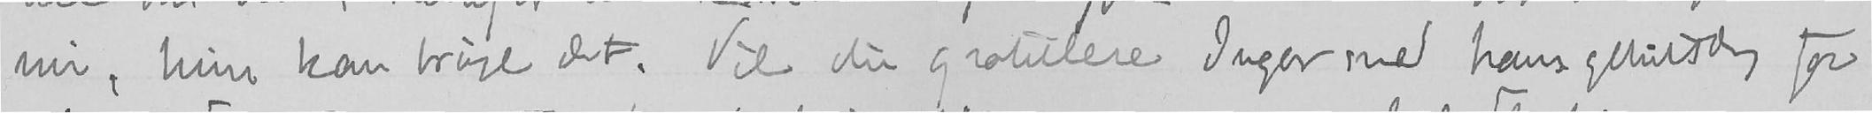nu, hun kan bruge det. Vil du gratulere Ingar med hans geburtsdag for-
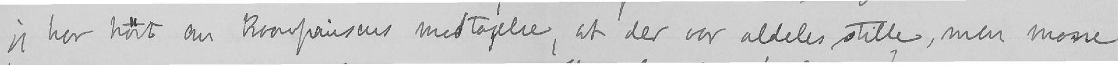jeg har hørt om Kronprinsens modtagelse, at der var aldeles stille, men masse
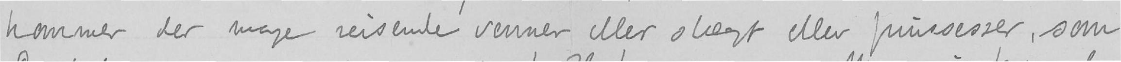kommer der mange reisende venner eller slægt eller prinsesser, som
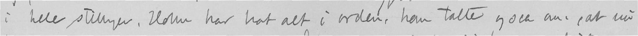i hele stillingen, Holm har hat alt i orden, han talte ogsaa om, at nu
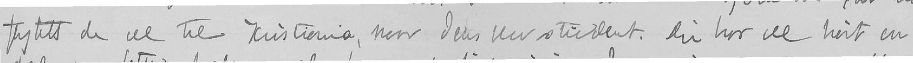flyttet de vel til Kristiania, naar Jens blev student. Du har vel hørt en
